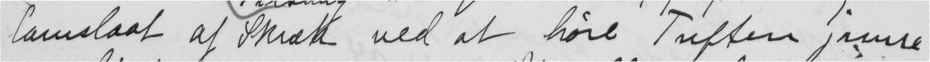lamslaat af Skræk ved at høre Tuften jamre
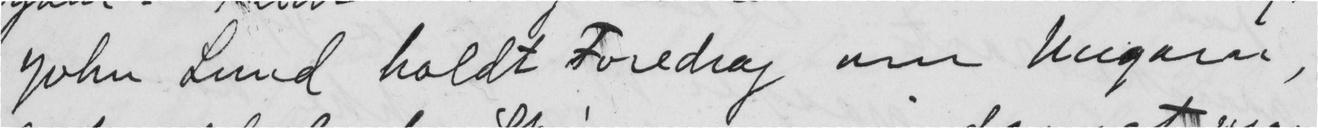Joln Lund holdt Foredrag om Ungarn,
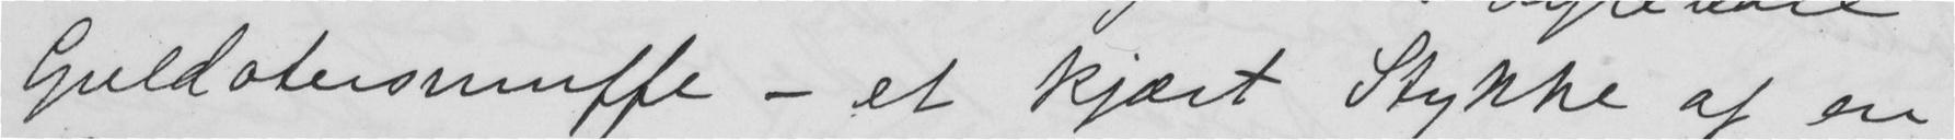Guldotersmuffe - et kjært Stykke af en
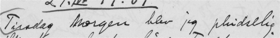Tirsdag Morgen blev jeg pludselig
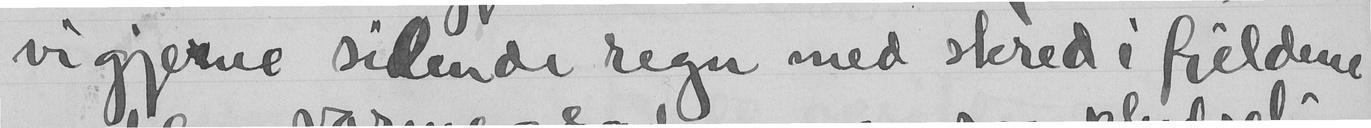vi gjerne silende regn med skred i fjeldene
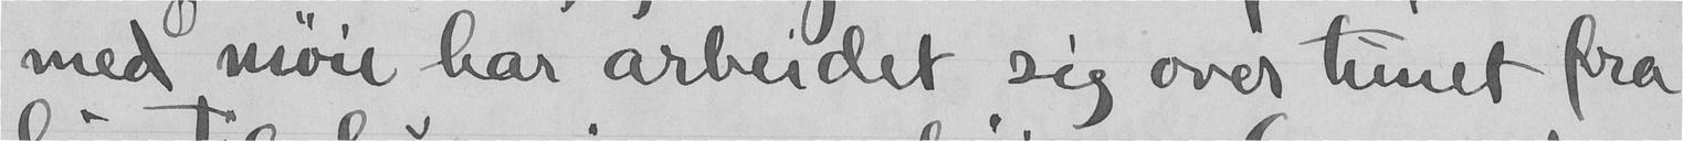med møie har arbeidet sig over tunet fra
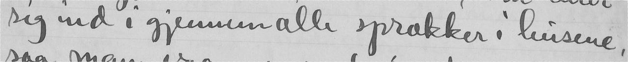sig ind i gjennem alle sprækker i husene,
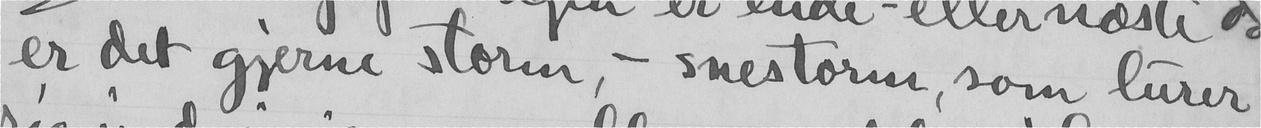er det gjerne storm, - snestorm som lurer
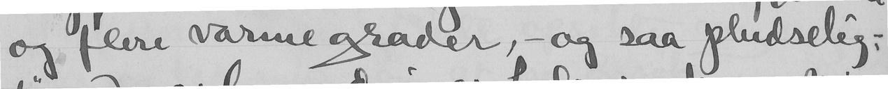og flere varme grader, - og saa pludselig:
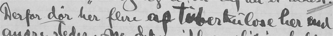Derfor dør her flere af toberkulose her end
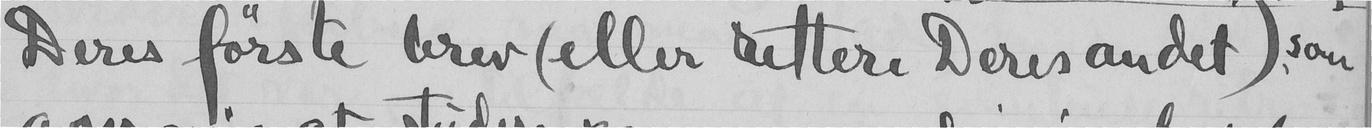Deres første brev (eller rettere Deres andet), som
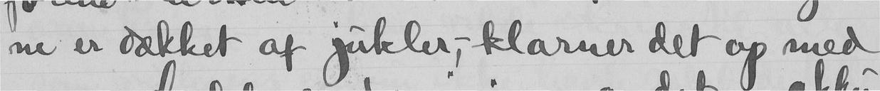ne er dækket af jukler, klarner det op med
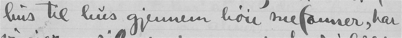hus til hus gjennem høie snefonner, har
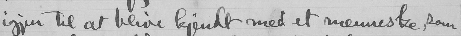igjen til at blive kjendt med et menneske, som
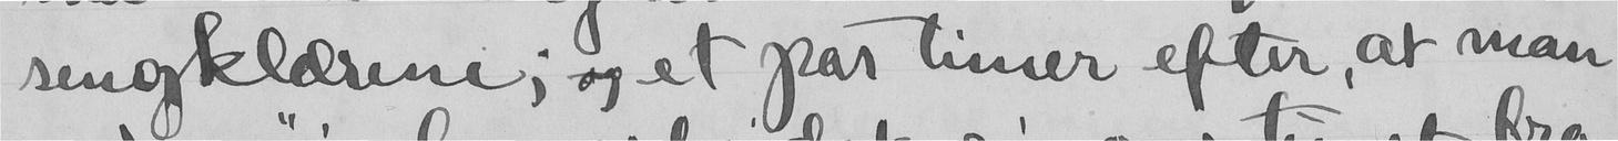sengklærene, og et par timer efter, at man
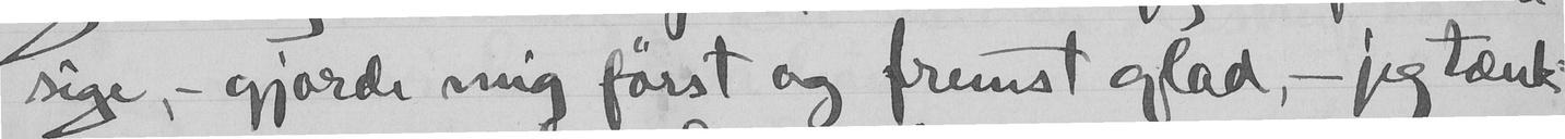sige, - gjorde mig først og fremst glad, - jeg tænk-
